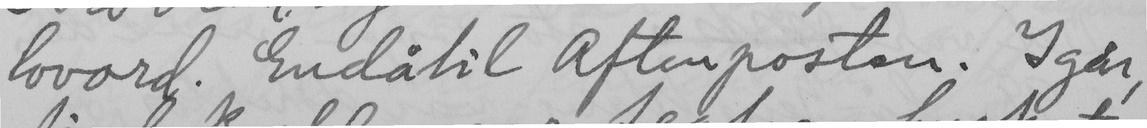lovord. Endåtil Aftenposten. Igår,
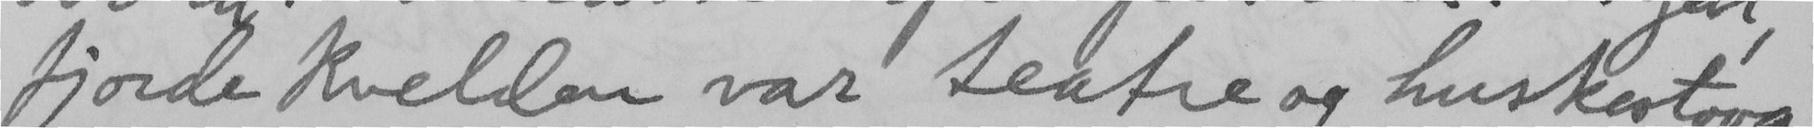fjorde kvelden var teatre og huskestova,
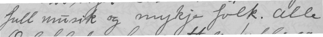full musik og mykje folk. Alle
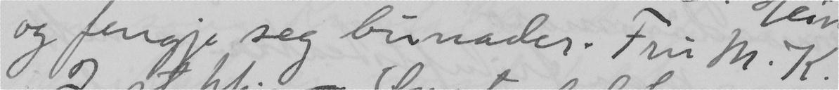og fengje seg bunader. Fru M.K.
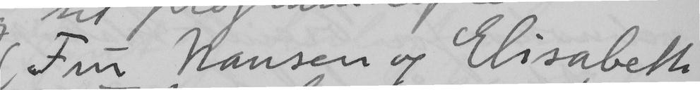Fru Nansen og Elisabeth
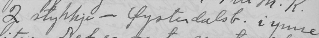2 stykkje – Öysterdalsb. i ymse
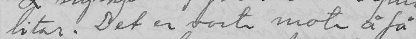litar. Det er vorte mote å få
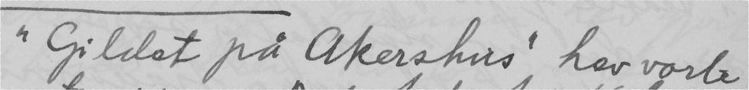"Gildet på Akershus" hev vorte
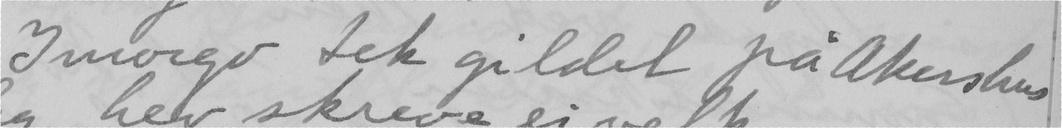Imorgo tek gildet på Akershus
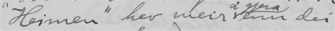"Heimen" hev meir å gjera enn dei
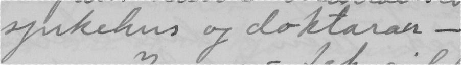sjukehus og doktarar –
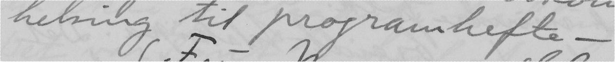helsing til programhefte –
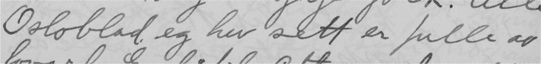Osloblad eg hev sett er fulle av
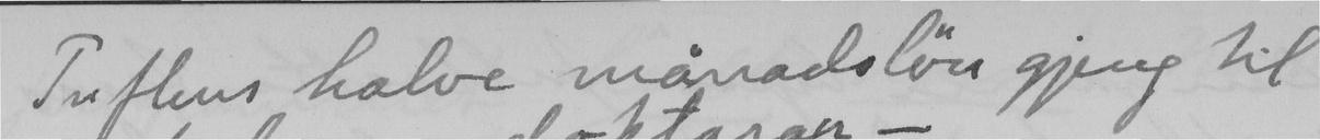Tuftens halve månadslön gjeng til
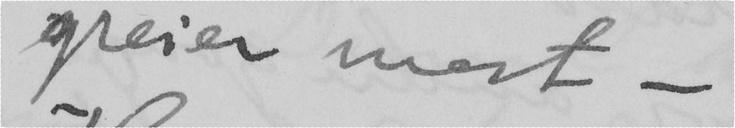greier mest –
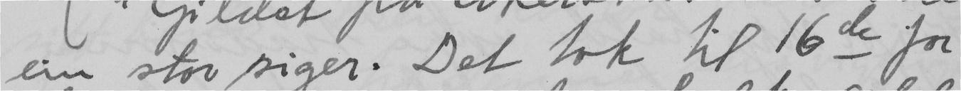ein stor siger. Det tok til 16de for
